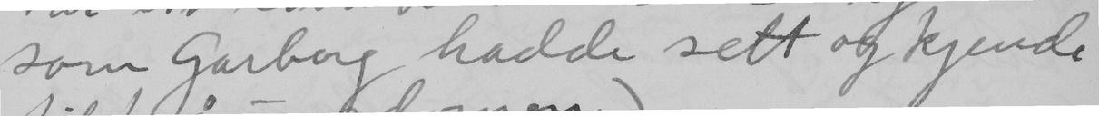som Garborg hadde sett og kjende
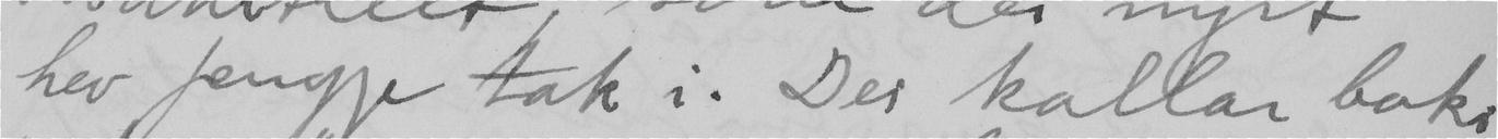hev fengje tak i. Dei kallar boki
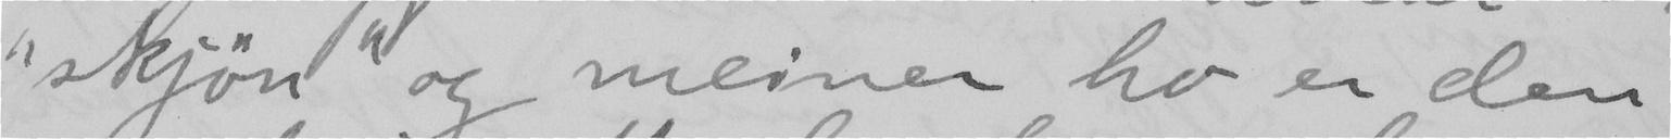"skjön" og meiner ho er den
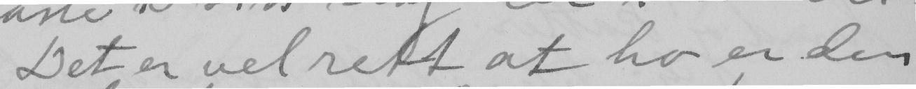Det er vel rett at ho er den
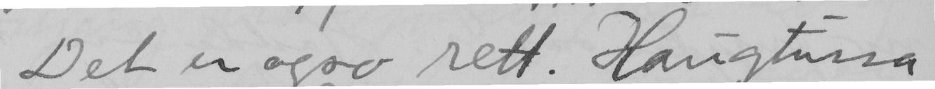Det er ogso rett. Haugtussa
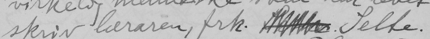skriv lesaren, frk.  Selte.
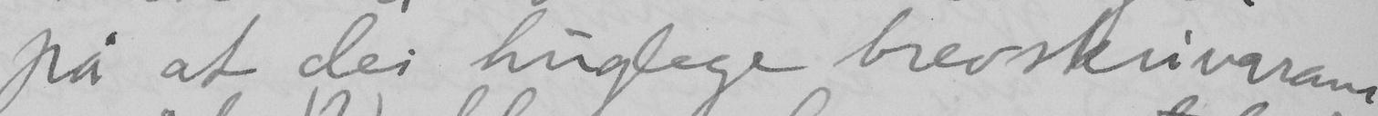på at dei huglege brevskrivarana
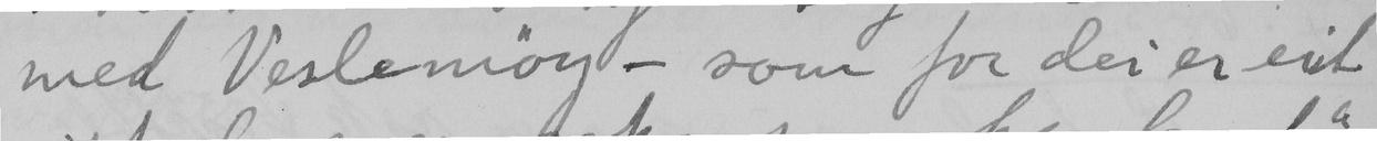med Veslemöy – som for dei er eit
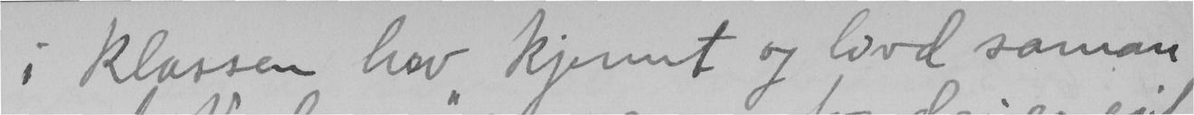i klassen hev kjennt og livd saman
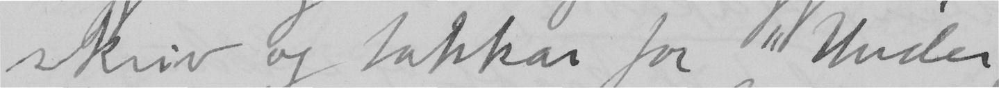skriv og takkar for "Under
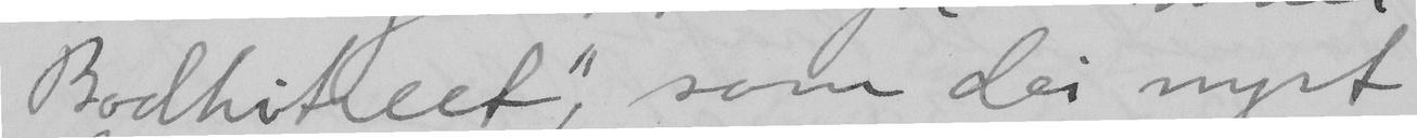Bodhitreet" som dei nyst
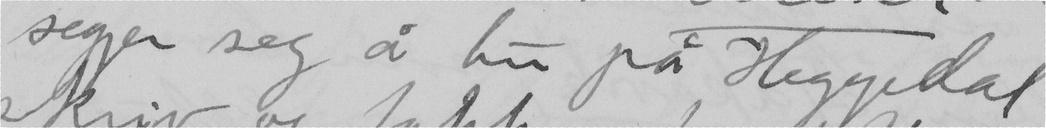segjer seg å bu på Heggedal
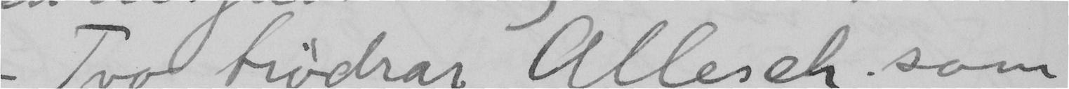Tvo brödrar Alleseh som
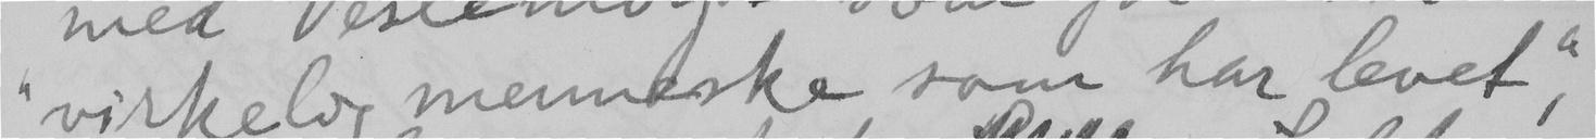"virkelig menneske som har levet",
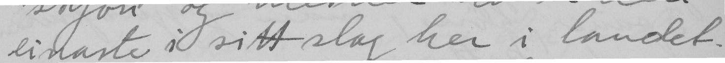einaste i sitt slag her i landet.
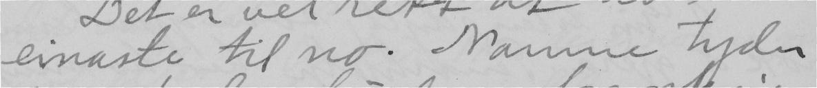einaste til no. Namne tyder
